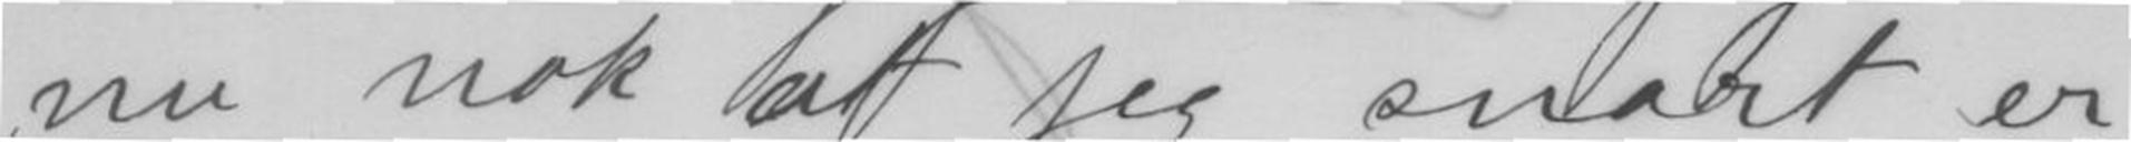nu nok at jeg snart er
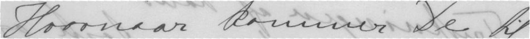Hvornaar kommer De til
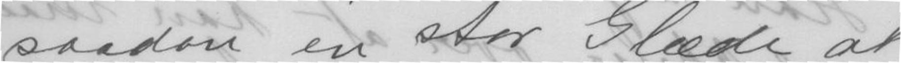saadan en stor Glæde at
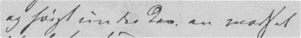og først under en modsat
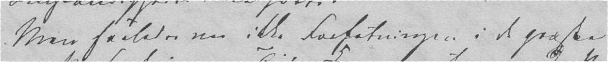Men saaledes var ikke Forfatningen i de græske
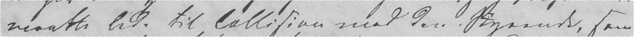maatte lede til Collision med den Styrende, som
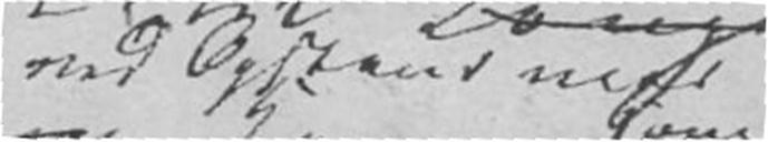ved Opstand mod
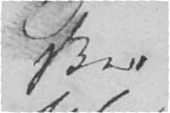sker
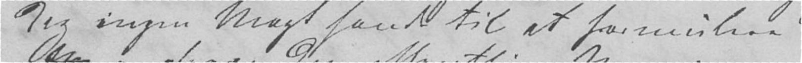dog ingen Magt havde til at formulere
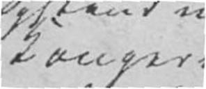Konger
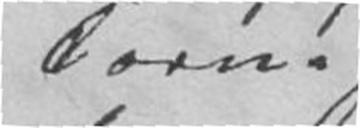Tarv.
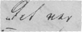Det var
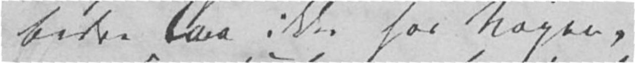bedre laa ikke hos Nogen,
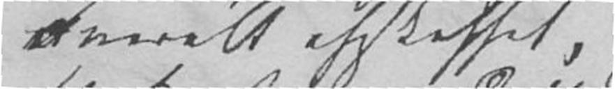overalt afskaffet,
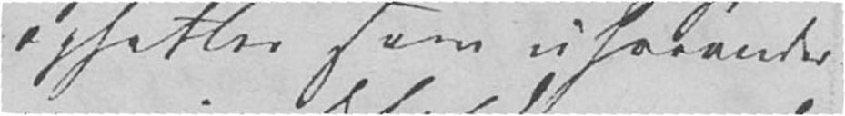opfattes som uforander-
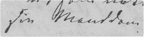sin Manddom
